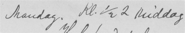Mandag. Kl. ½ 2 Middag
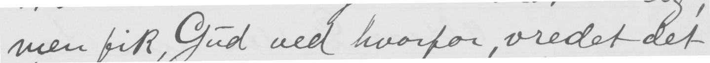men fik, Gud ved hvorfor, vredet det
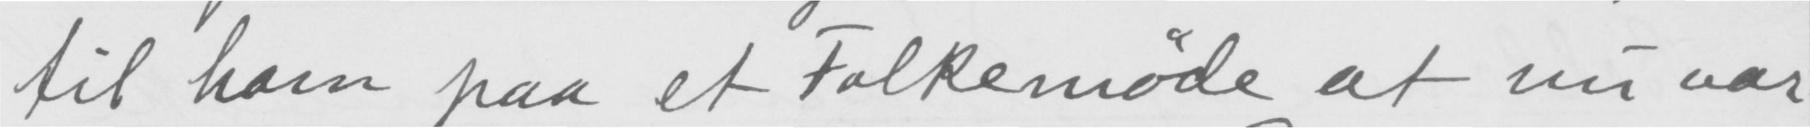til ham paa et Folkemøde at nu var
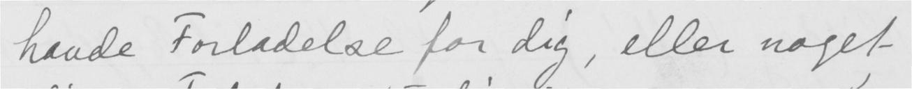havde Forladelse for dig, eller noget
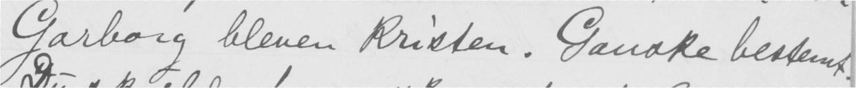Garborg bleven Kristen. Ganske bestemt.
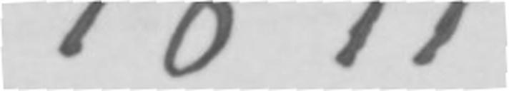1891
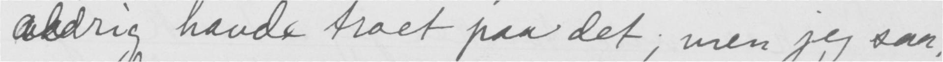aldrig havde troet paa det; men jeg saa,
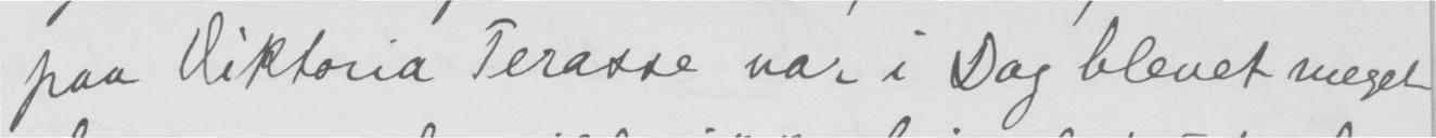paa Viktoria Terasse var i Dag blevet meget
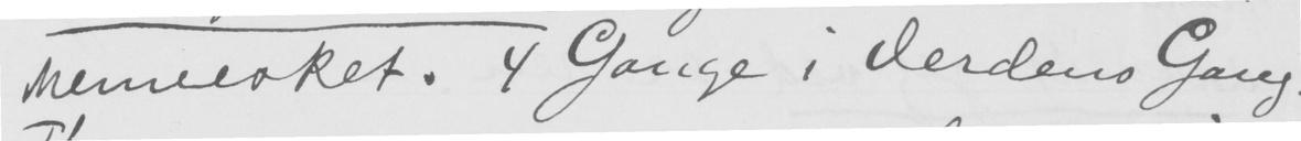Mennesket. 4 Gange i Verdens Gang.
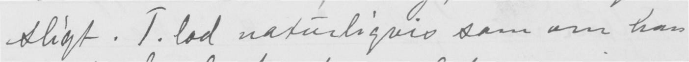sligt. T. lod naturligvis som om han
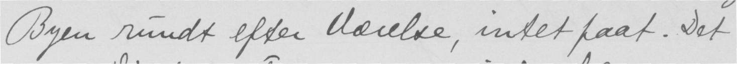Byen rundt efter Værelse, intet faat. Det
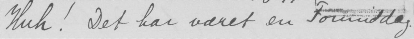Huh! Det har været en Formiddag
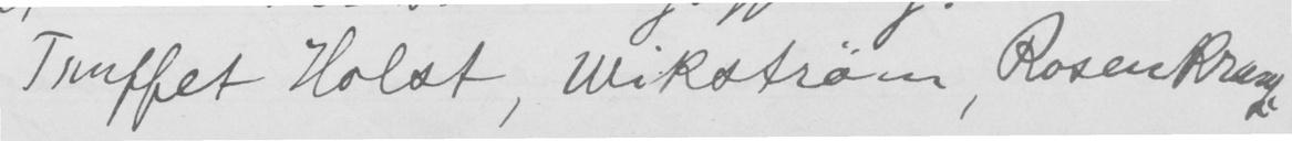Truffet Holst, Wikstrøm, Rosenkrag,
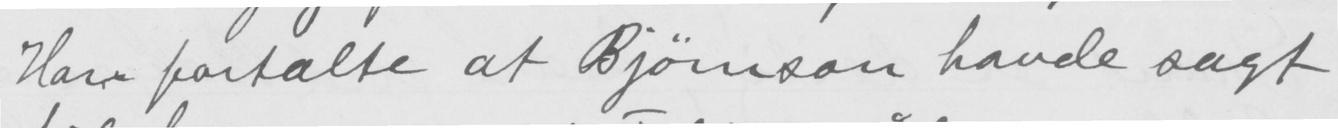Han fortalte at Bjørnson havde sagt
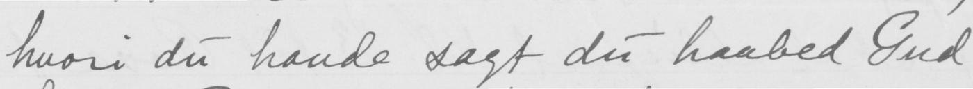hvori du havde sagt du haabed Gud
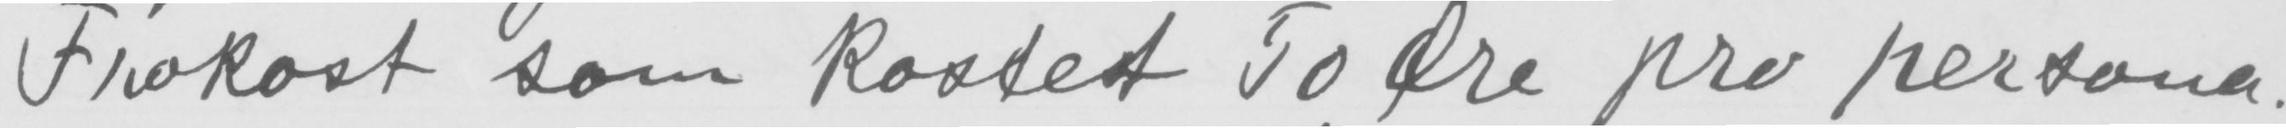Frokost som kostet 50 Øre pro persona.
